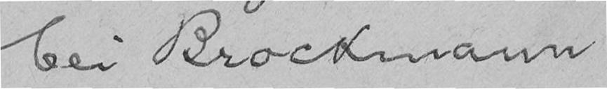bei Brockmann
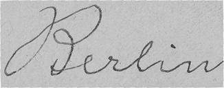Berlin
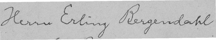Herrn Erling Bergendahl
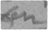en
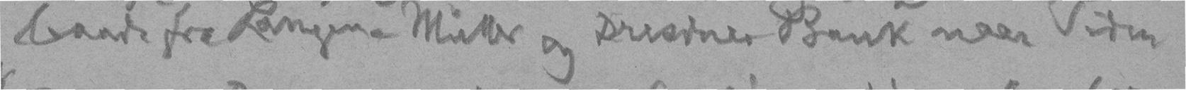baade fra Langen-Müller og Dresdner Bank naar Tiden
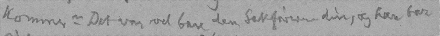kommer? Det var vel bare den Sakføreren din, og han bare
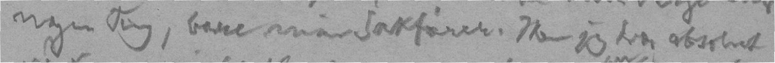nogen Ting, bare min Sakfører. Men jeg tror absolut
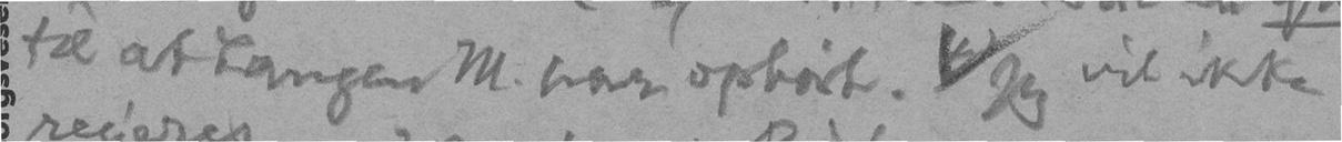til at Langen M. har Ophørt. 4) Jeg vil ikke
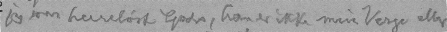jeg var herreløst Gods, han er ikke min Verge eller
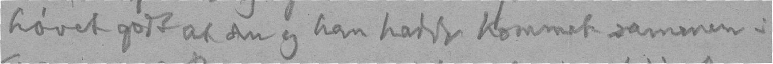høvet godt at du og han hadde kommet sammen i
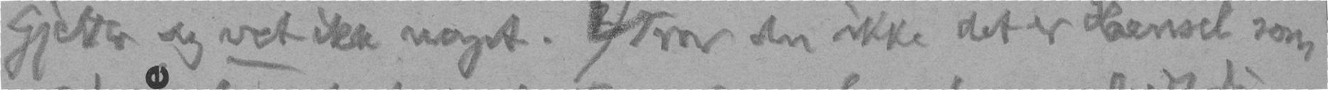gjetter og vet ikke noget. 2) Tror du ikke det er Haensel som
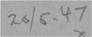20/5. 47
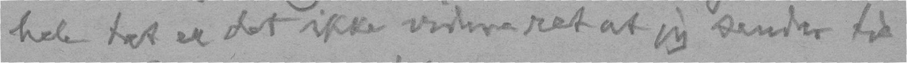hele tat er det ikke videre ret at jeg sender til
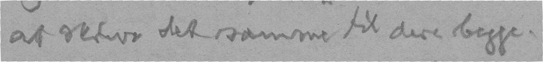at skrive det samme til dere begge.
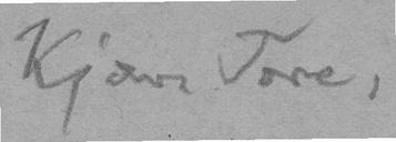Kjære Tore,
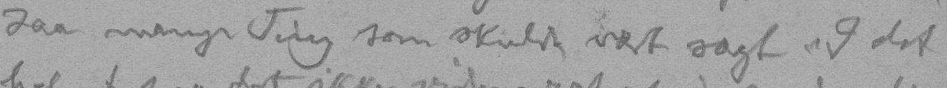saa mange Ting som skulde vært sagt. I det
